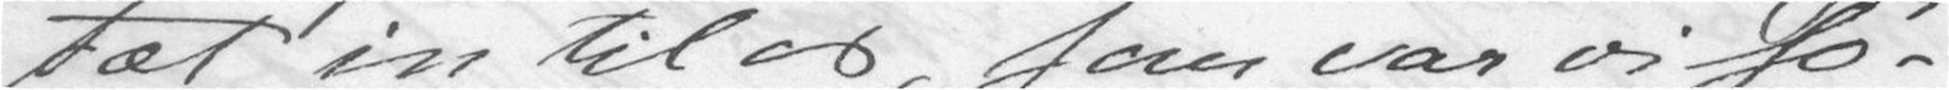tæt in til os, som var vi sø-
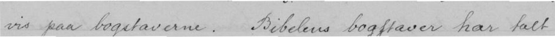vis paa bogstaverne. Bibelens bogstaver har talt
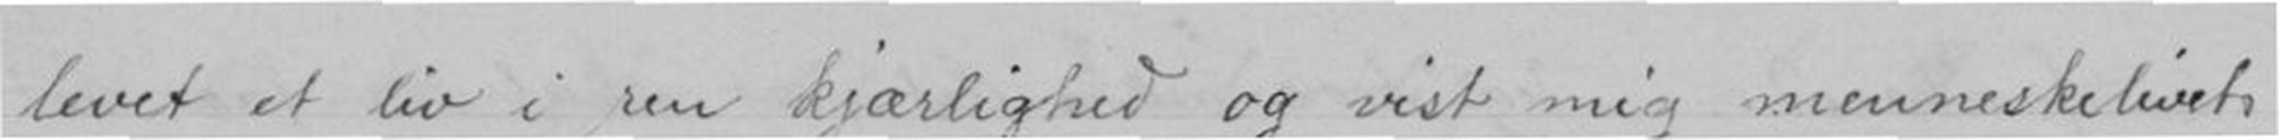levet et liv i ren kjærlighed og vist mig menneskelivets
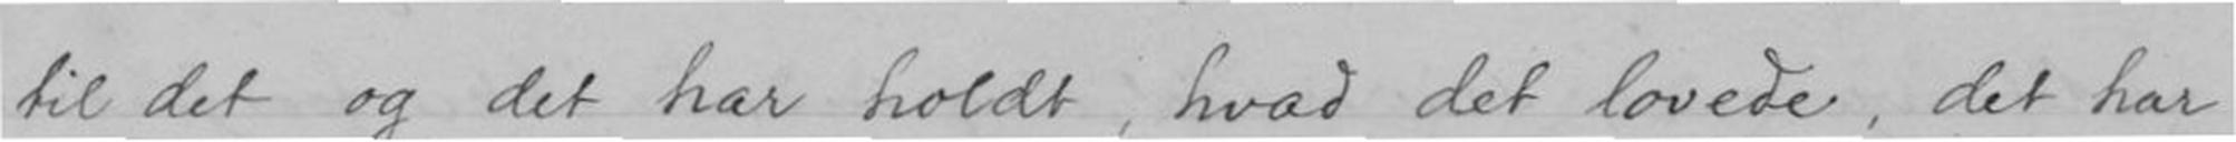til det og det har holdt hvad det lovede, det har
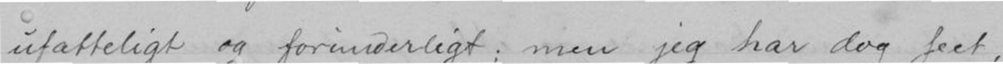ufatteligt og forundrerligt; men jeg har dog seet,
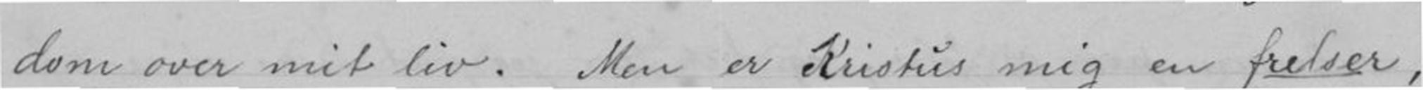dom over mit liv. Men er Kristus mig en frelser,
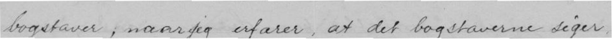bogstaver; naar jeg erfarer, at det bogstaverne siger
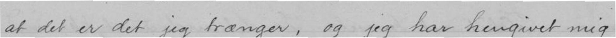at det er det jeg trænger, og jeg har hengivet mig
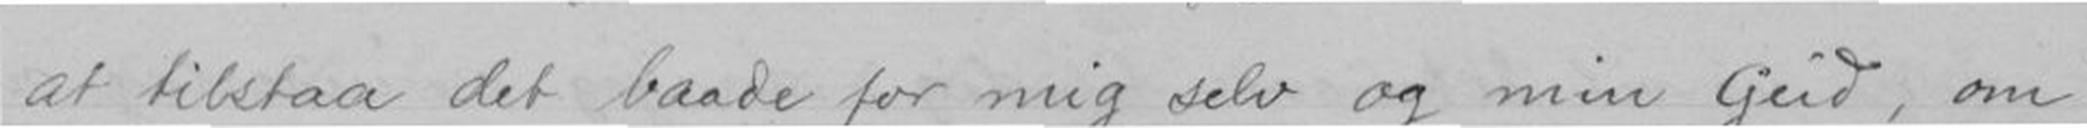at tilstaa det baade for mig selv og min Gud, om
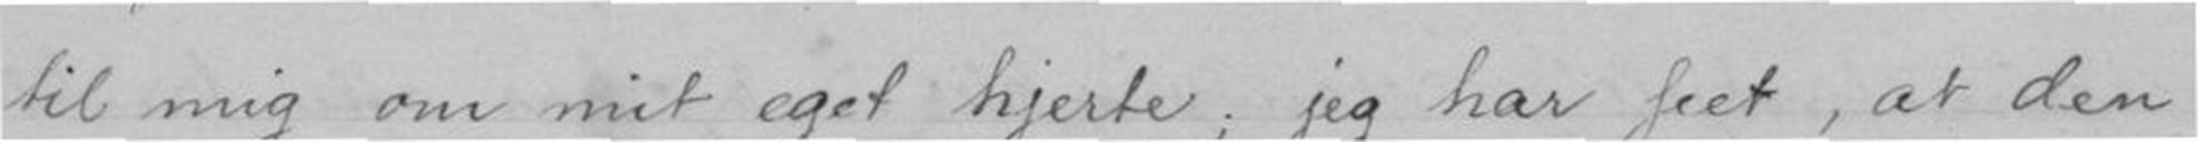til mig om mit eget hjerte; jeg har seet, at den
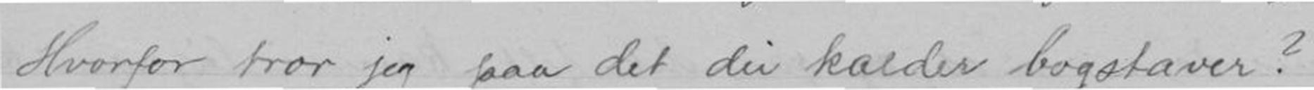Hvorfor tror jeg paa det du kalder bogstaver?
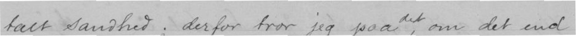talt sandhed; derfor tror jeg paa det, om det end
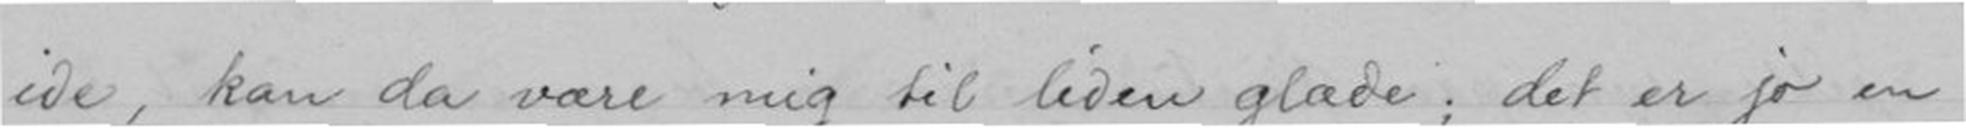ide, kan da være mig til liden glæde; det er jo en
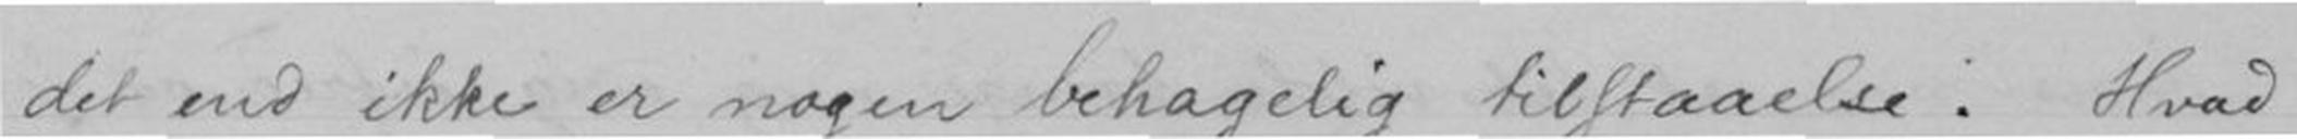det end ikke er nogen behagelig tilstaaelse. Hvad
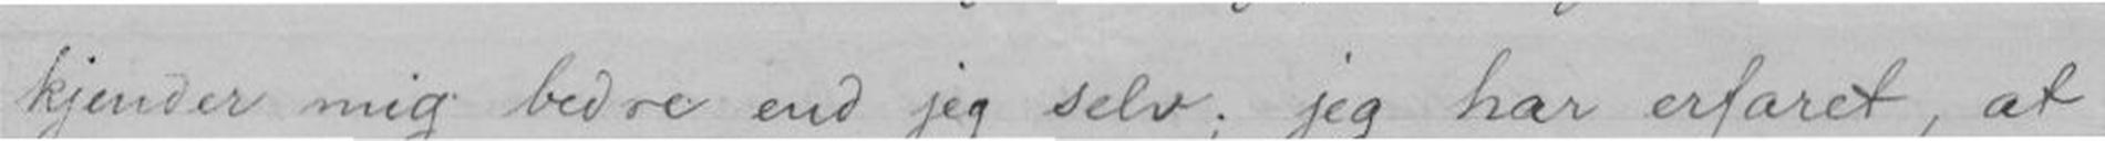kjender mig bedre end jeg selv; jeg har erfaret, at
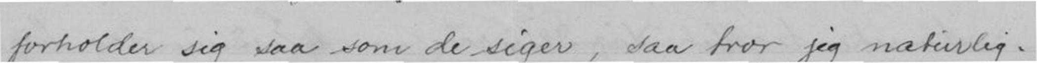forholder sig saa som de siger, saa tror jeg naturlig-
